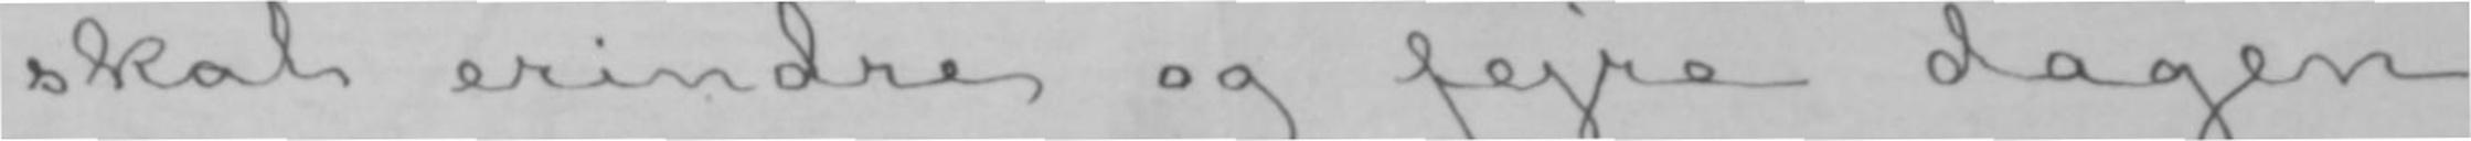skal erindre og fejre dagen
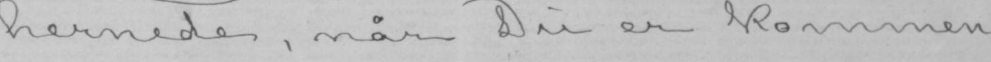hernede, når Du er kommen
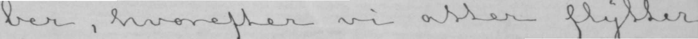ber, hvorefter vi atter flytter
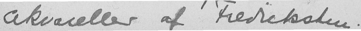akvareller af Fredriksten.
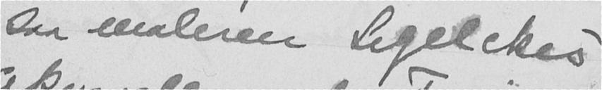saa maleren Segelckes
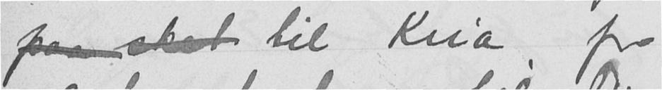paa sket til Kria, for
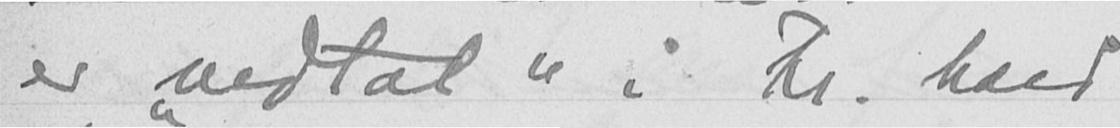er "vedtat" i Fr. hald
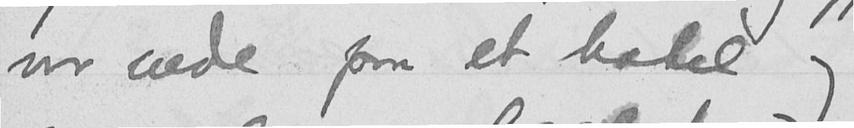var nede paa et hotel og
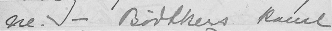ne. - Bødtkers kanal
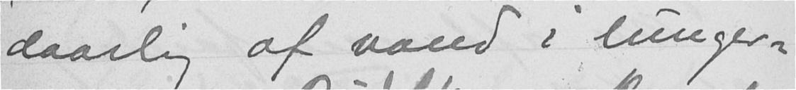daarlig af vand i lunger-
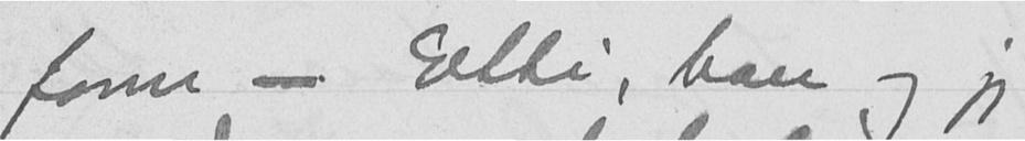form - Etti, han og jeg
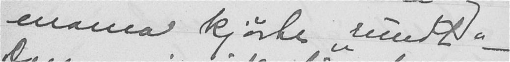mama kjørte "rundt" -
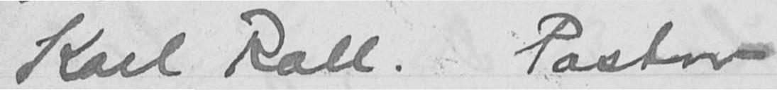Karl Roll. Pastor
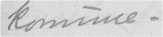komune.
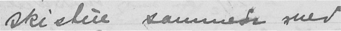skistue sammen med
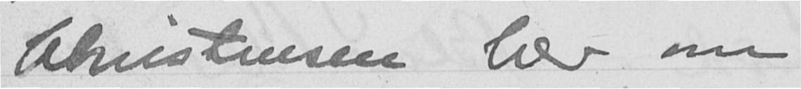Christensen her om
